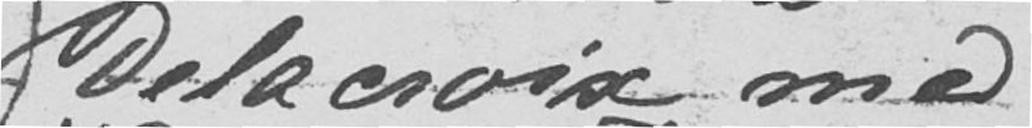Delacroix med
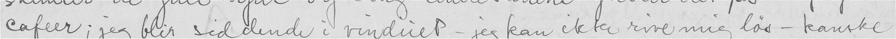cafeer; jeg blir siddende i vinduet - jeg kan ikke rive mig løs - kanske
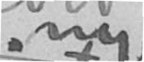nye
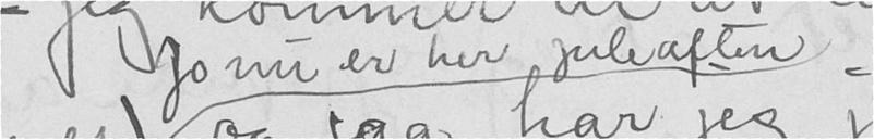jo nu er her juleaften
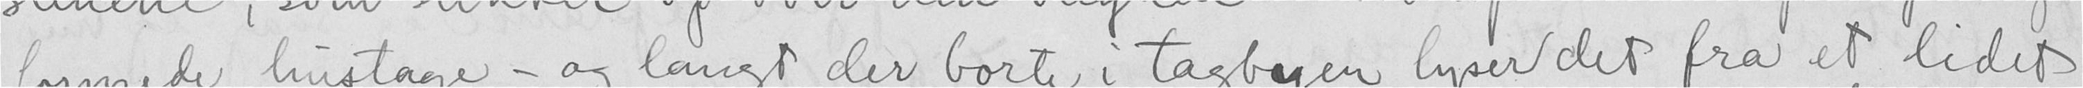formede hustage - og langt der borte i tagbyen lyser det fra et lidet
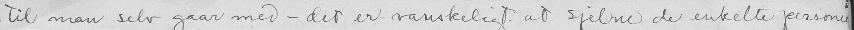til man selv gaar med - det er vanskeligt at sjelne de enkelte personer
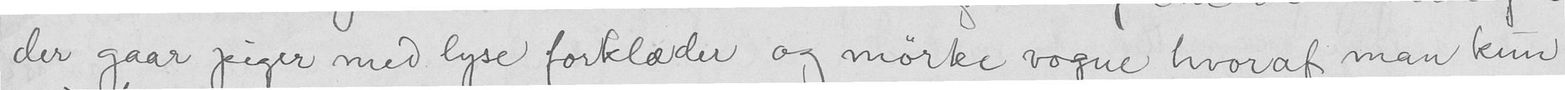der gaar piger med lyse forklæder og mørke vogne hvoraf man kun
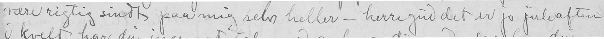være rigtig sindt paa mig selv heller - herregud det er jo juleaften
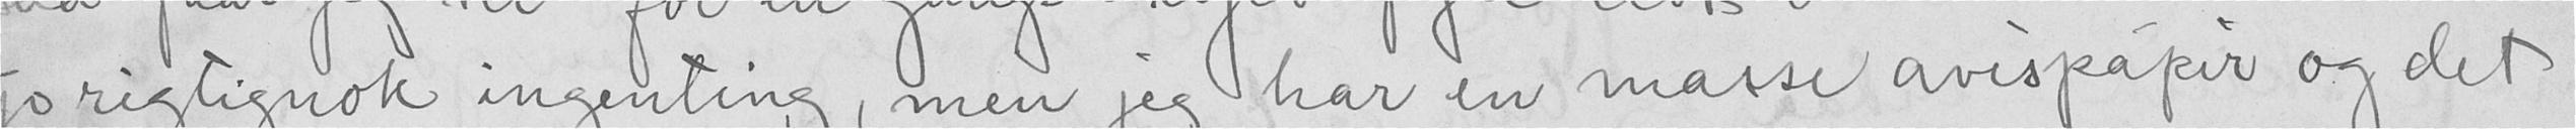jo rigtignok ingenting, men jeg har en masse avispapir og det
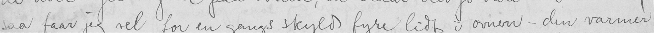saa faar jeg vel for en gangs skyldd fyre lidt i ovnen - den varmer
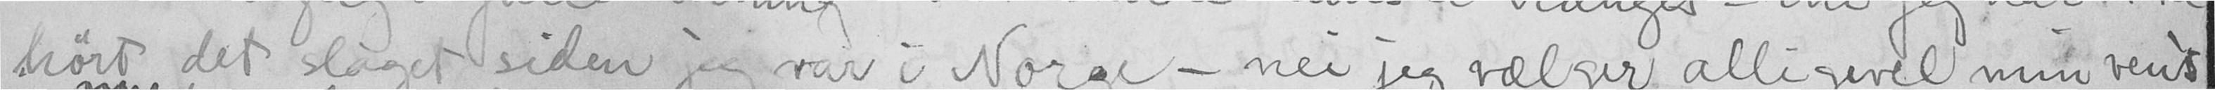hørt det slaget siden jeg var i Norge - nei jeg vælger alligevel min vens
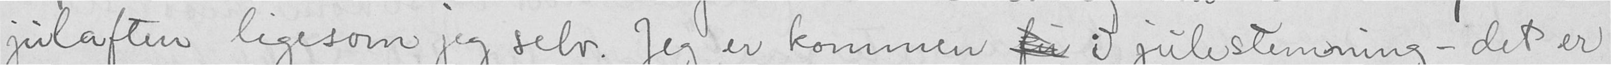julaften ligesom jeg selv. Jeg er kommer  i julestemning - det er
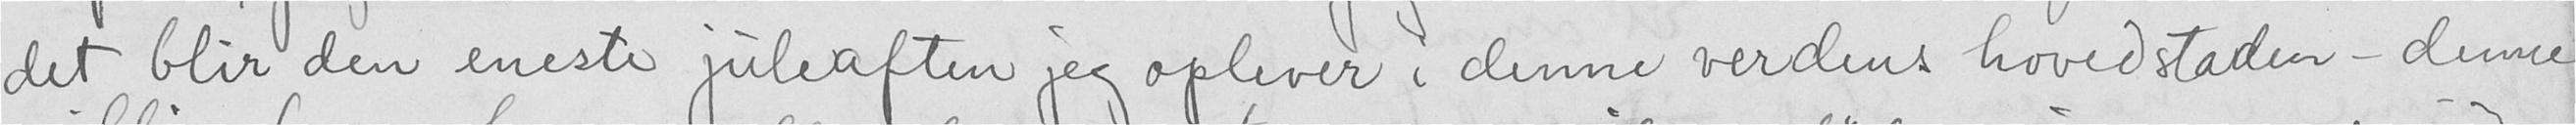det blir den eneste juleaften jeg oplever i denne verdens hovedstaden - denne
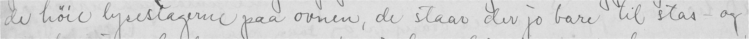de høie lysestagerne paa ovnen, de staar der jo bare til stas - og
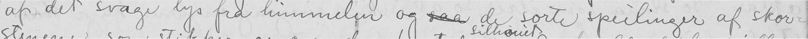af det svage lys fra himmelen og  de sorte speilinger af skor-
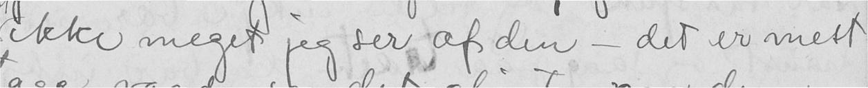ikke meget jeg ser af den - det er mest
